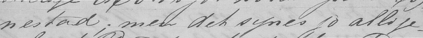nestad; men det synes jo allige-
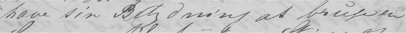have sin Betydning at bruge en
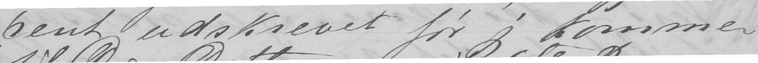rent udskrevet, før jeg kommer
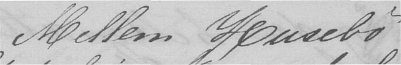Mellem Husebø
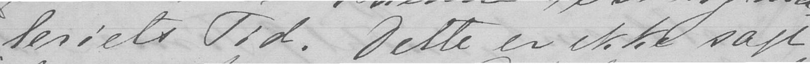leriets Tid. Dette er ikke sagt
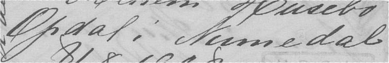Opdal i Numedal
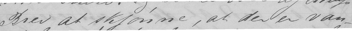Brev at skjønne, at der er van-
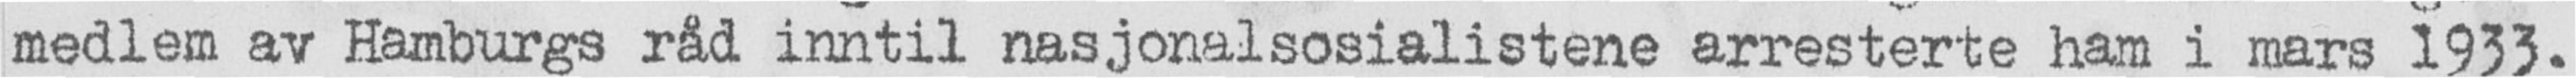medlem av Hamburgs råd inntil nasjonalsosialistene arresterte ham i mars 1933.
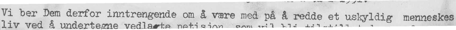Vi ber Dem derfor inntrengende om å være med på å redde et uskyldig menneskes
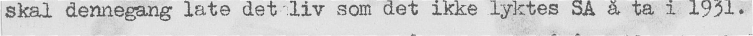skal dennegang late det liv som det ikke lyktes SA å ta i 1931.
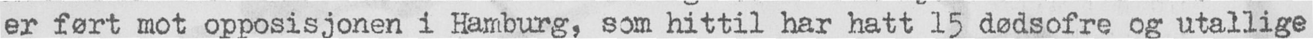er ført mot opposisjonen i Hamburg, som hittil har hatt 15 dødsofre og utallige
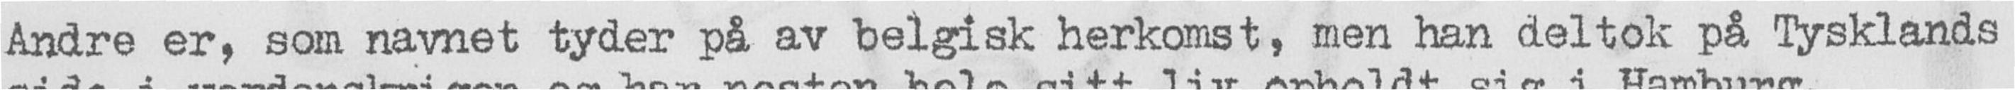Andre er, som navnet tyder på av belgisk herkomst, men han deltok på Tysklands
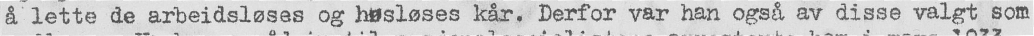å lette de arbeidsløses og husløses kår. Derfor var han også av disse valgt som
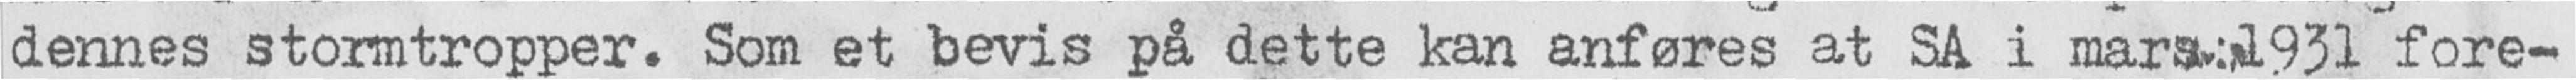dennes stormtropper. Som et bevis på dette kan anføres at SA i mars 1931 fore-
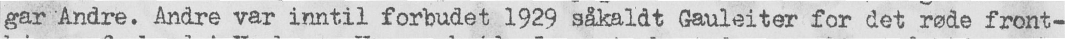gar Andre. Andre var inntil forbudet 1929 såkaldt Gauleiter for det røde front-
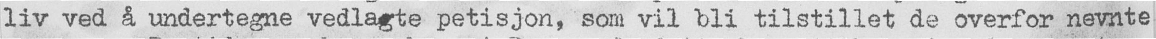liv ved å undertegne vedlagte petisjon, som vil bli tilstillet de overfor nevnte
